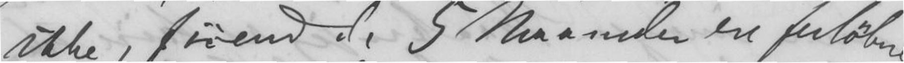ikke, førend de 5 Maaneder ere forløbne
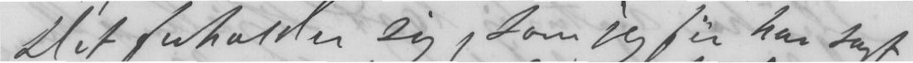Det forholder sig, som jeg før har sagt
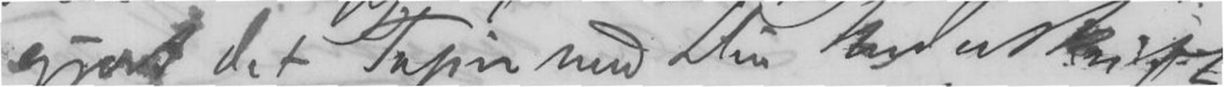gjort det Papir med Din Underskrift,
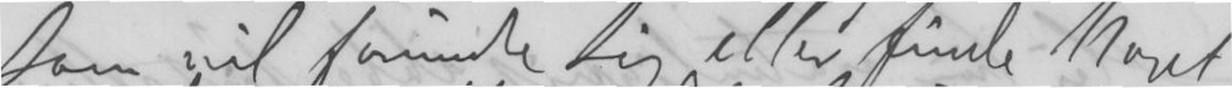som vil forundre sig eller finde Noget
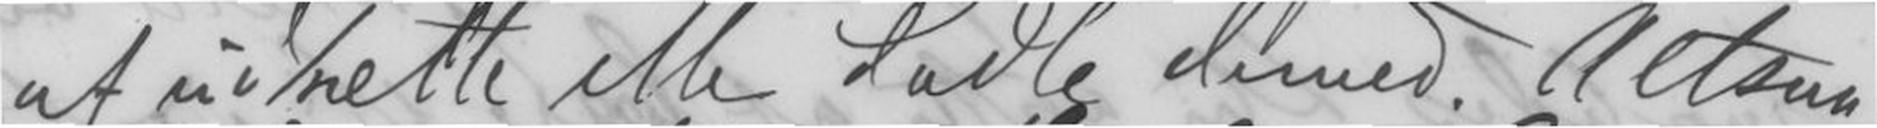at udrette eller Dadle derved. Altsaa
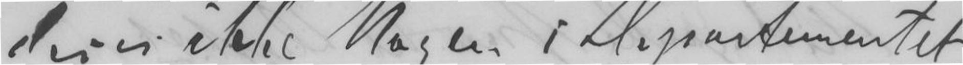der er ikke Nogen i Departementet
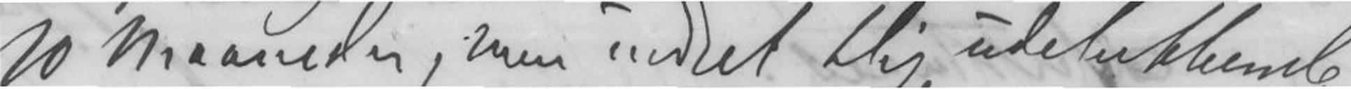10 Maaneder, men indret Dig udelukkende
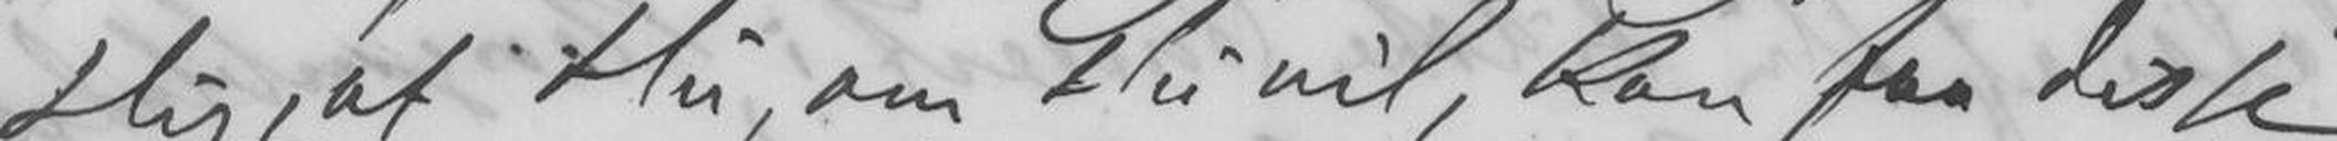Dig, at Du, om Du vil, kan faa disse
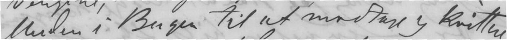Anden i Bergen til at modtage og kvittere
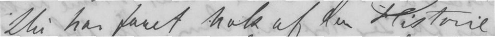Du har faaet nok af den Historie,
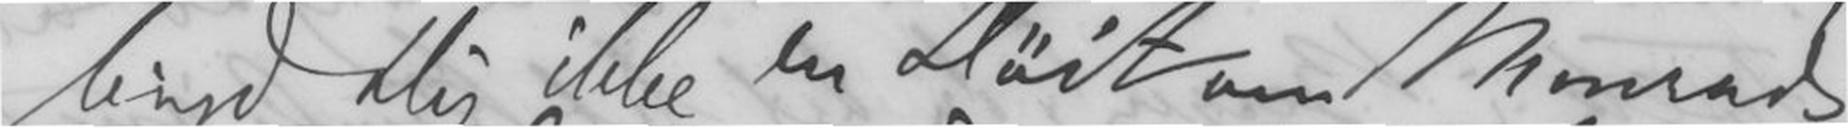bryd Dig ikke en Døit om Monrads
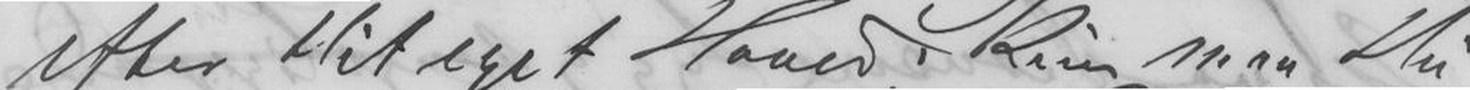efter Dit eget Hoved. Kun maa Du
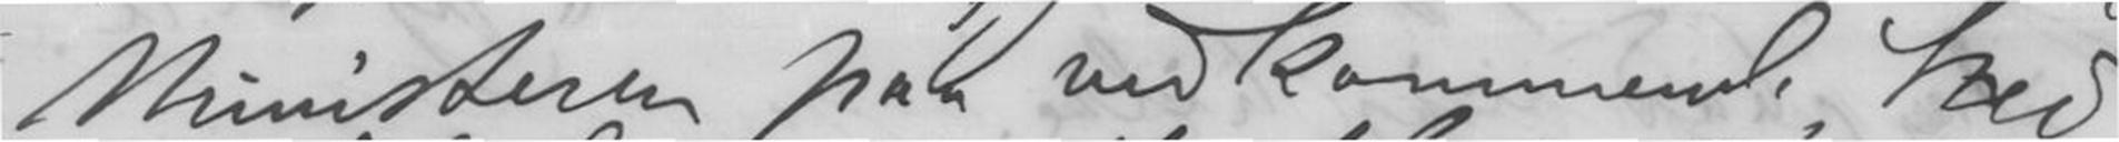Ministeren paa vedkommende Sted
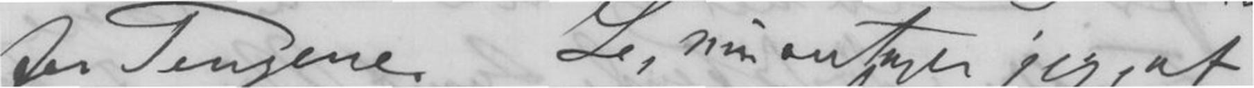for Pengene. Se, nu antager jeg, at
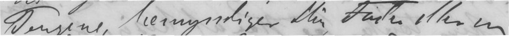Pengene, bemyndiger Din Fader eller en
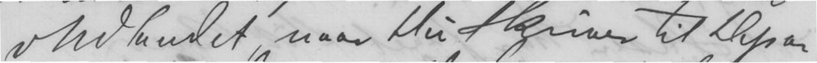v/Udlandet, naar Du skriver til Depar-
